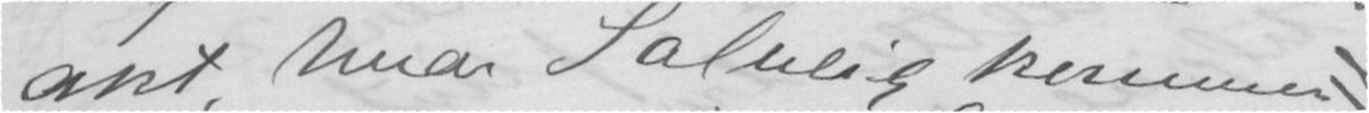akt, hvor Solveig kommer
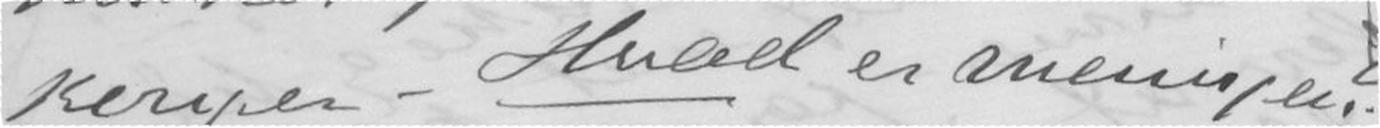Keri.er - Hvad er meningen?
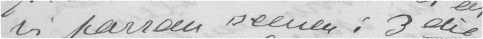vi forran scenen i 3die
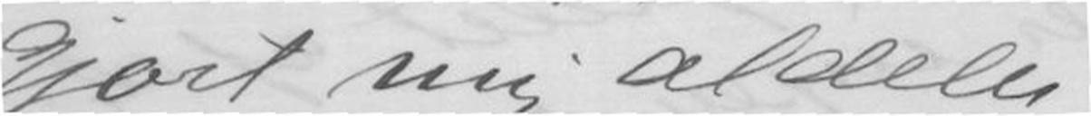gjort mig aldeles
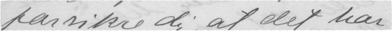forsikre dig, at det har
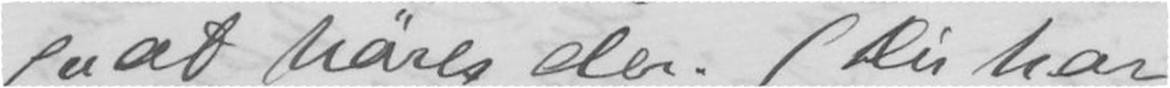gaat høres der. (Du har
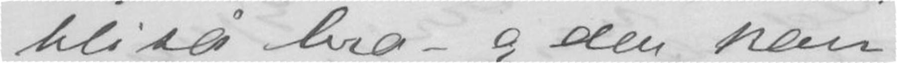bli så bra - og den kan
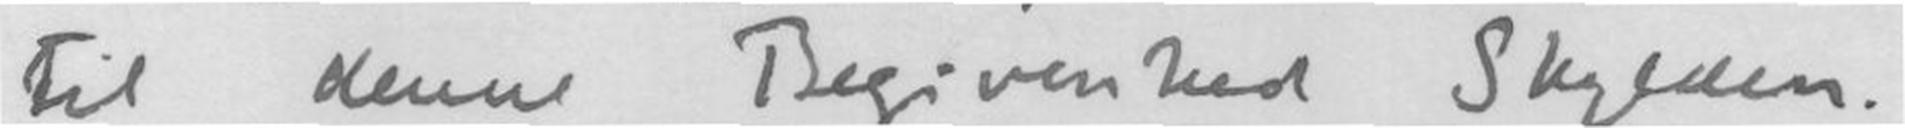til denne Begivenhed Skylden.
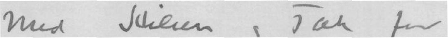Med Hilsen og Tak for
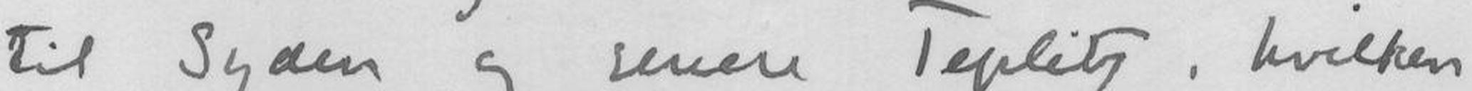til Syden og senere Teplitz, hvilken
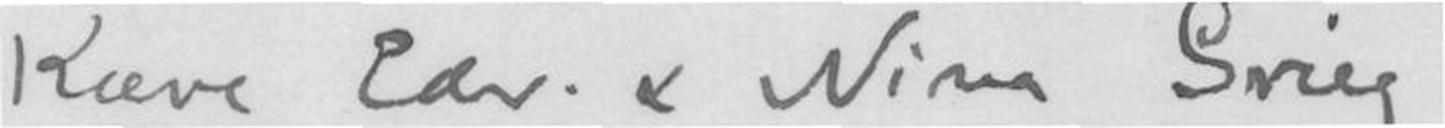Kære Edv. & Nina Grieg.
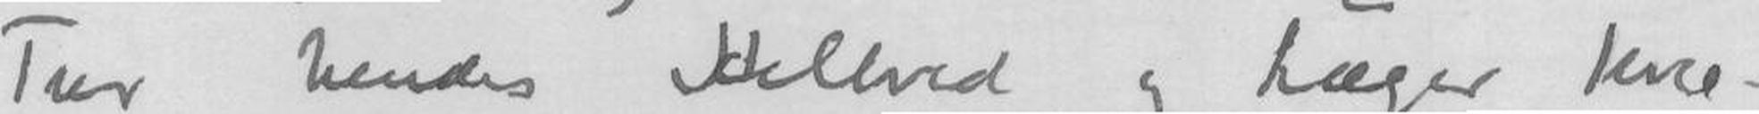Tur hendes Helbred og læger kræ-
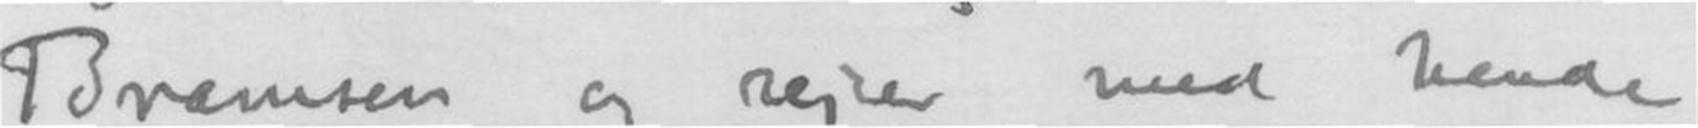Bramsen og rejser med hende
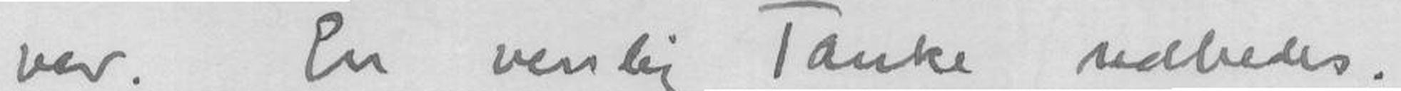ver. En venlig Tanke udbedes.
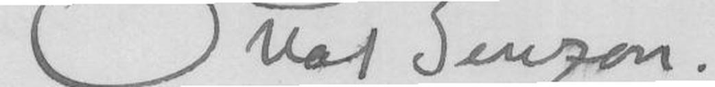Otto Benzon.
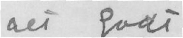alt godt
